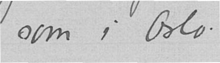som i Oslo.
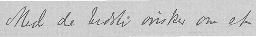Med de bedste ønsker om et
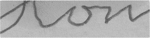Høn
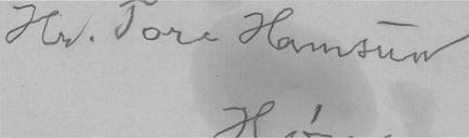Hr. Tore Hamsun
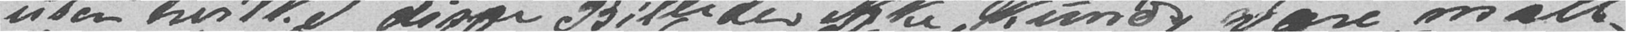uten hvilke disse Billeder ikke kunde være malt
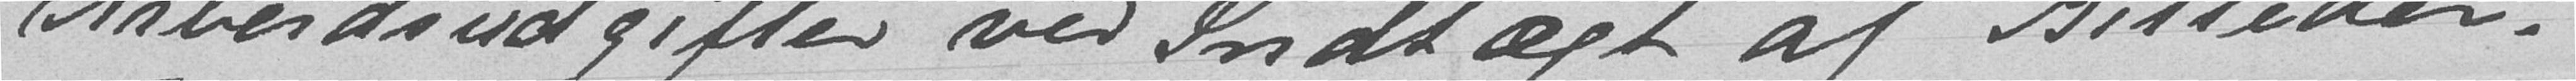Arbeidsudgifter ved Indtægt af Billeder
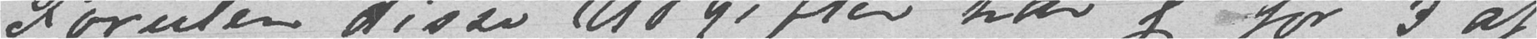Foruten disse Udgifter har jeg for 3 af
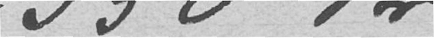350 kr
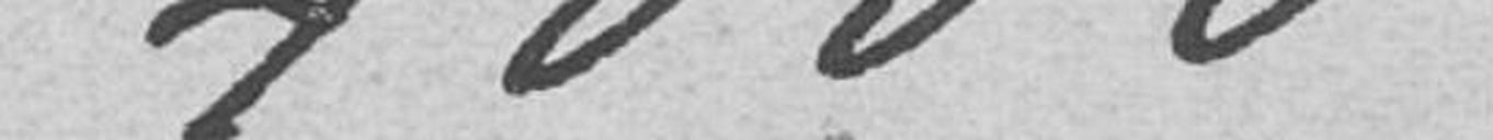4000
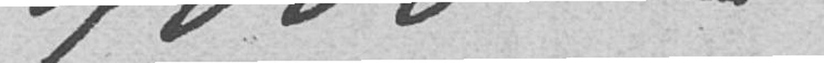4000
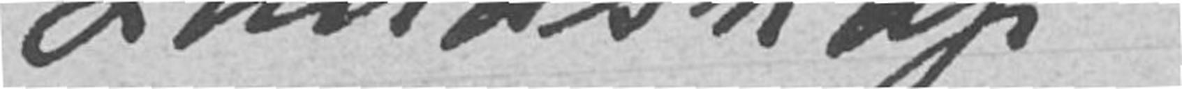Landskap
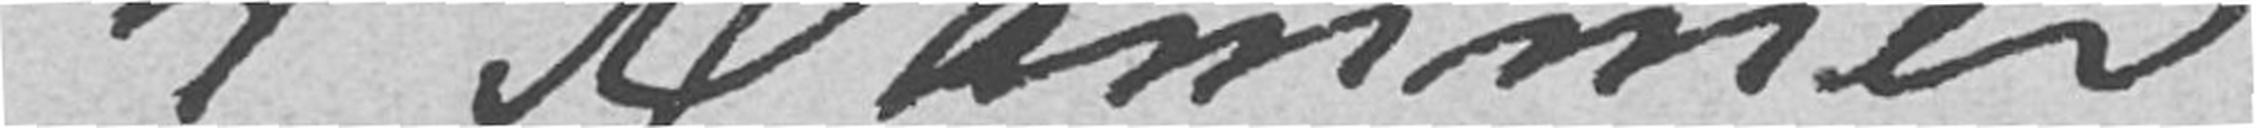4 Rammer
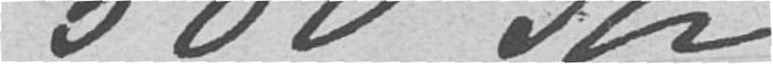500 Kr
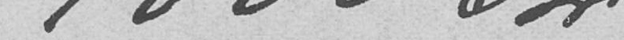1000 Kr
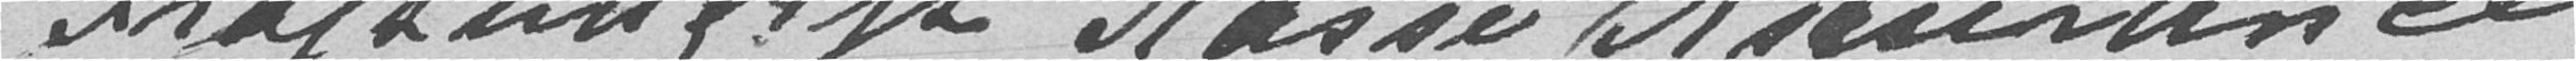Fragtudguft Kasse Assurance
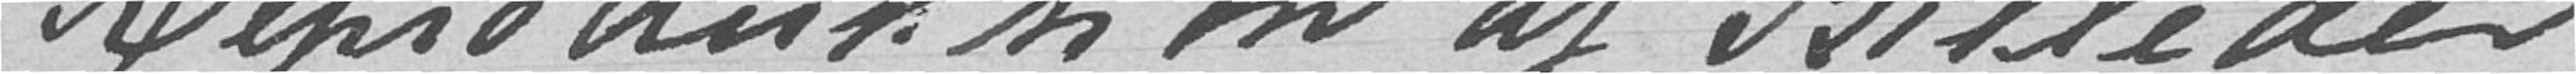Reproduktion af Billeder
